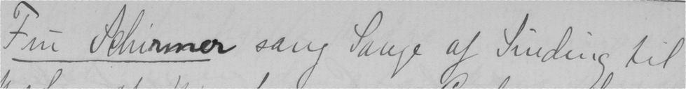Fru Schirmer sang Sange af Sinding til
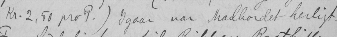Kr. 2, 50 pro P.) Igaar var Madbordet herligt.
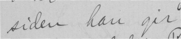siden han gir
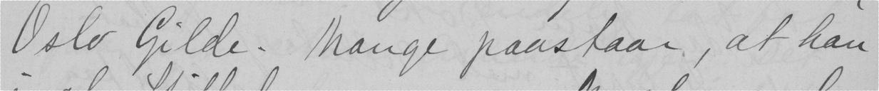Osto Gilde. Mange paastaar, at han
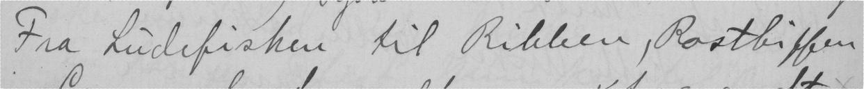Fra Ludefisken til Ribben, Rostbiffen
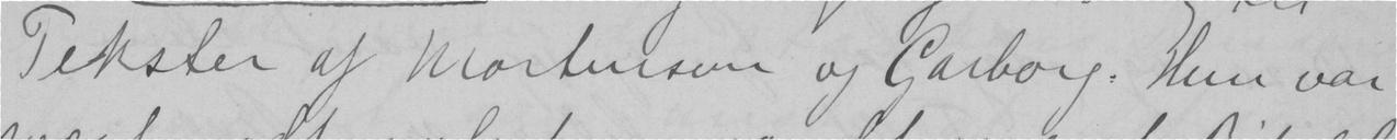Tekster af Mortenson og Garborg. Hun var
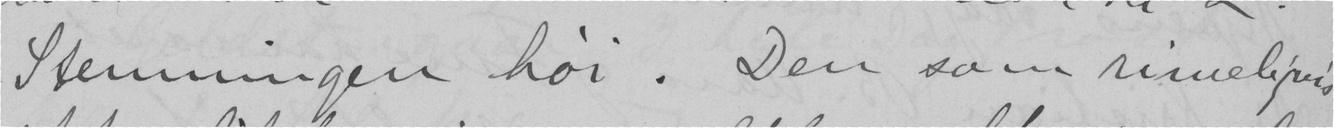Stemningen høi. Den som rimeligvis
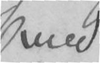med
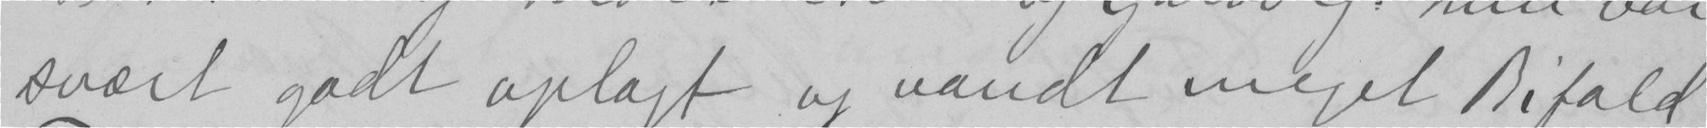svært godt oplagt og vandt meget Bifald
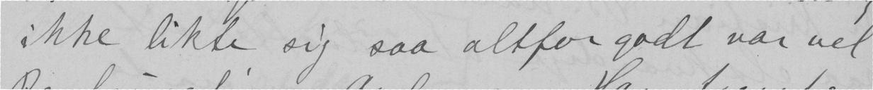ikke likte sig saa altfor godt var vel
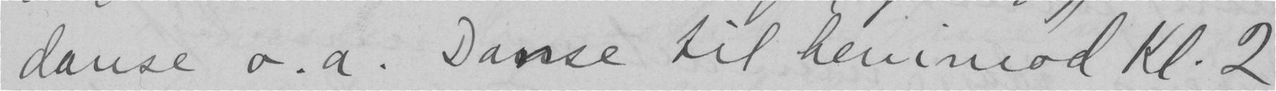danse o. a. Danse til henimod kl. 2.
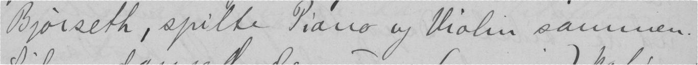Bjørseth, spilte Piano og Violin sammen.
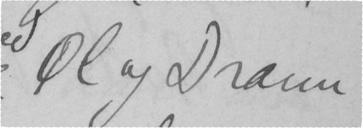Øl og Dram
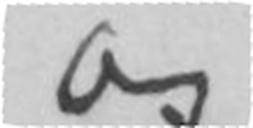os
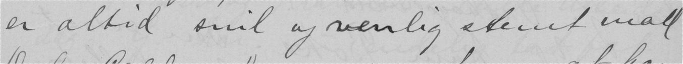er altid snil og venlig stemt mod
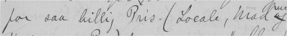for saa billig Pris. (Locale, Mad og
